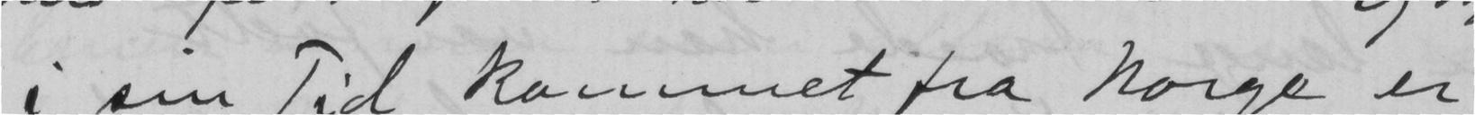i sin Tid kommet fra Norge er
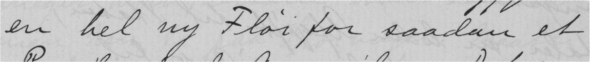en hel ny Fløi for saadan et
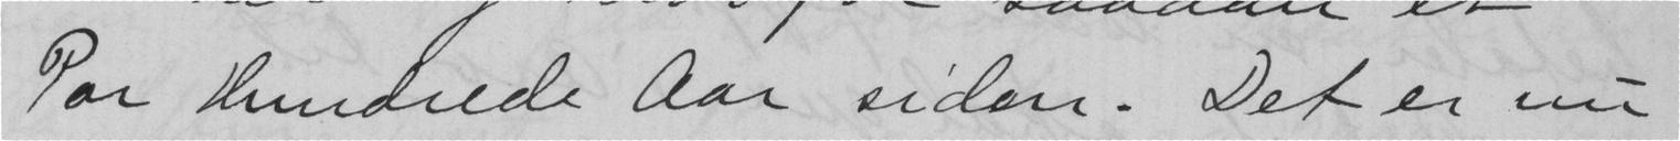Par Hundrede aar siden. Det er nu
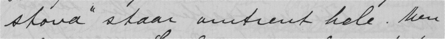stova" staar omtrent hele. Men
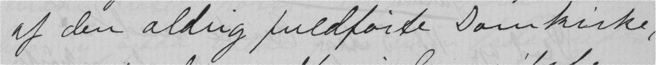af den aldrig fuldførte Domkirke,
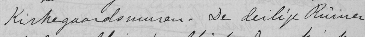Kirkegaardsmuren. De deilige Ruiner
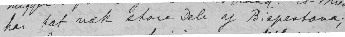har tat væk store Dele af Bispestova;
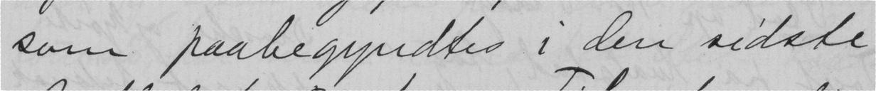som paabegyndtes i den sidste
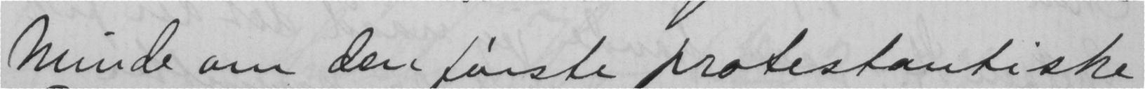Minde om den første protestantiske
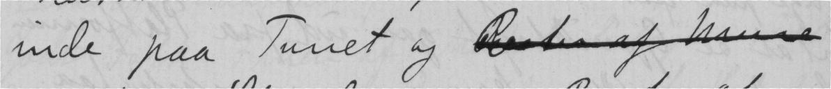inde paa Tunet og
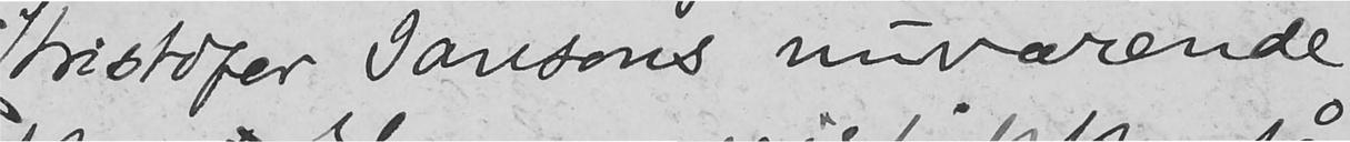Kristofer Jansons nuværende
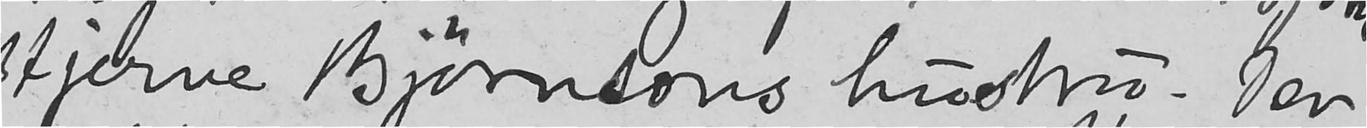tjerne Bjørnsons hustru. Der
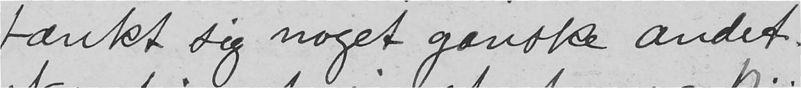tænkt sig noget ganske andet.
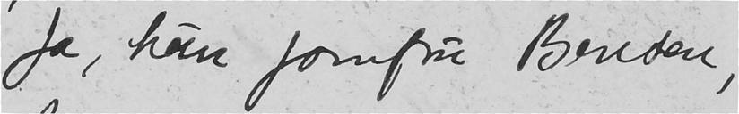ja, hun jomfru Bensen,
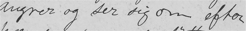angrer og ser sig om efter
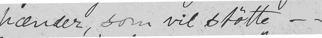hænder, som vil støtte --
